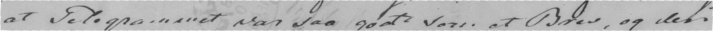at Telegrammet var saa godt som et Brev, og der i
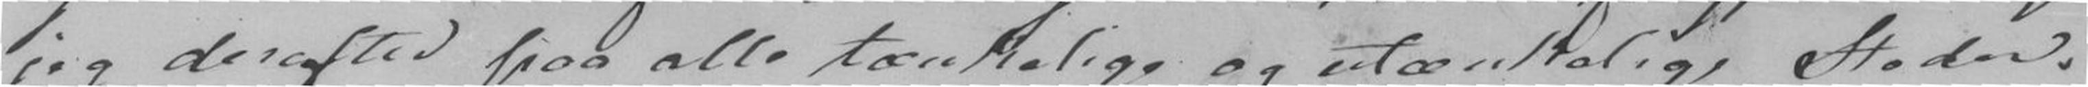jeg derefter paa alle tænkelige og utænkelige Steder.
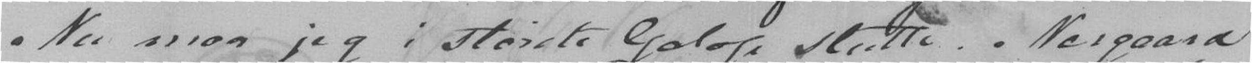Nu maa jeg i største Galop slutte. Nergaard
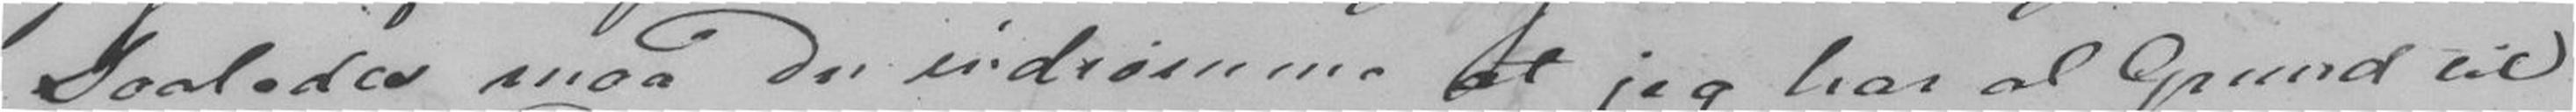Saaledes maa Du indrømme at jeg har al Grund til
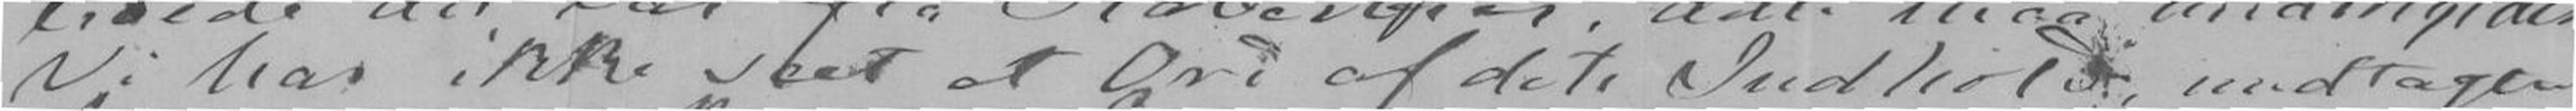Vi har ikke seet et Ord af dets Indhold, undtagen
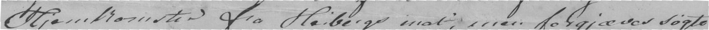Hjemkomsten fra Heibergs inat, men forgjæves søgte
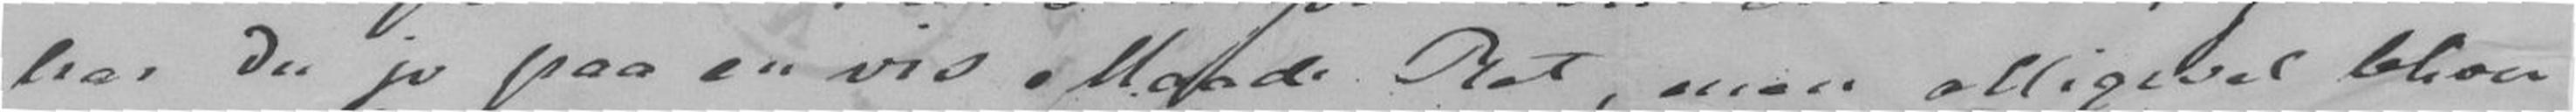har Du jo paa en vis Maade Ret, men alligevel bliver
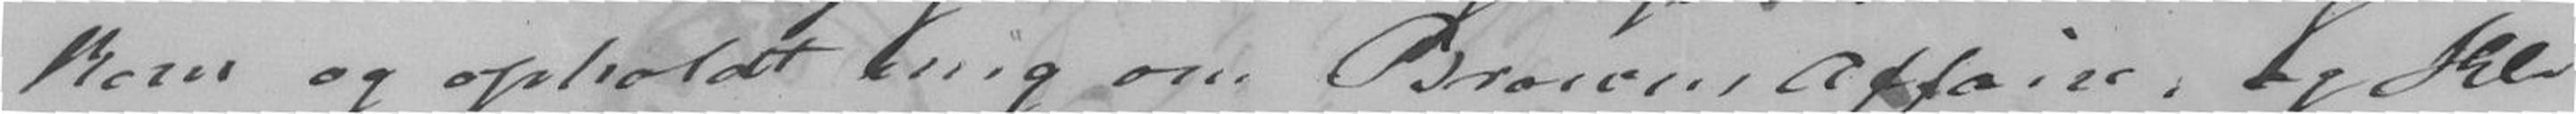kom og opholdt mig om Browns Affaire, og Kl.
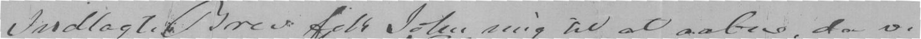Indlagte Brev fik John mig til at aabne, da vi
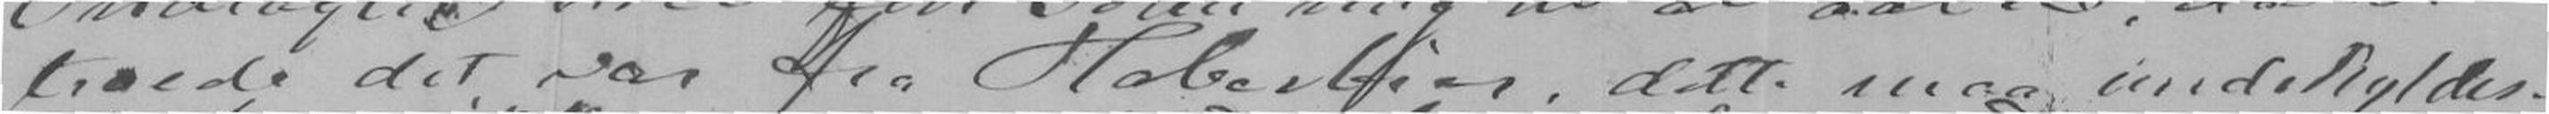troede det var fra Haberbier, dette maa undskyldes.
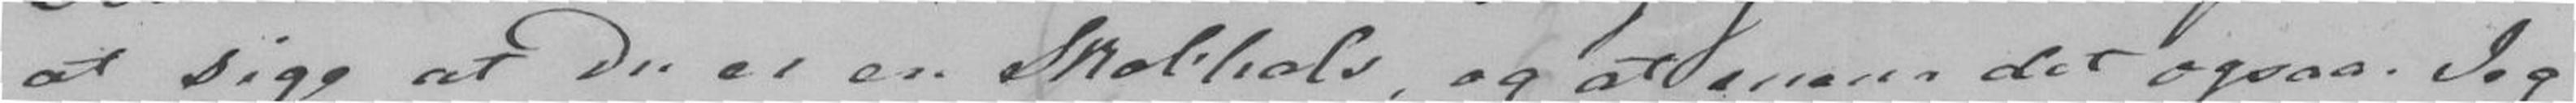at sige at Du er en Skabhals, og at mene det ogsaa. Jeg
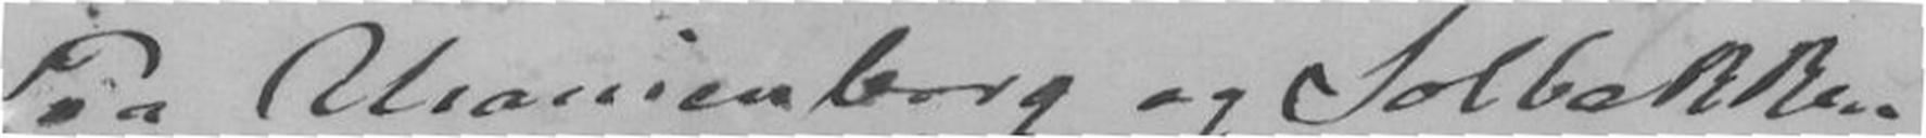Paa Uranienborg og Solbakken
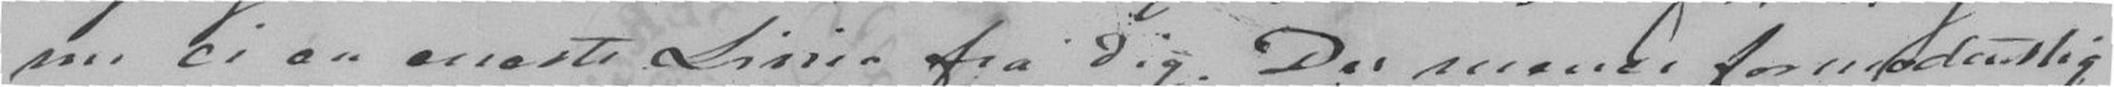nu ei en eneste Linie fra Dig. Du mener formodentlig
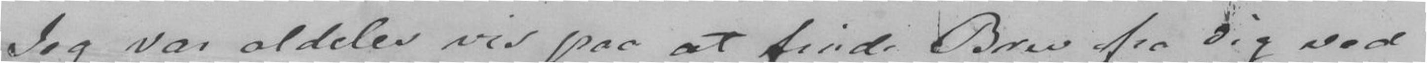Jeg var aldeles vis paa at finde Brev fra Dig ved
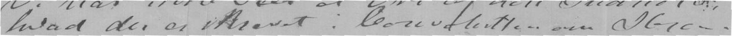hvad der er skrevet i Convolutten om Ibsen.
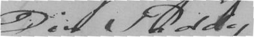Din Paddy
